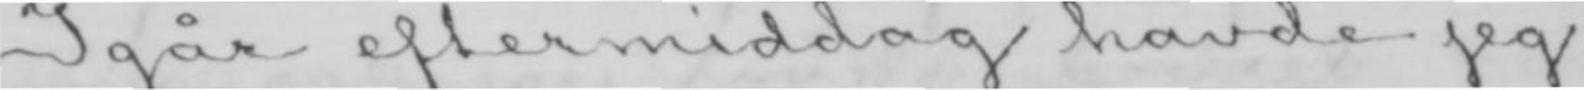Igår eftermiddag havde jeg
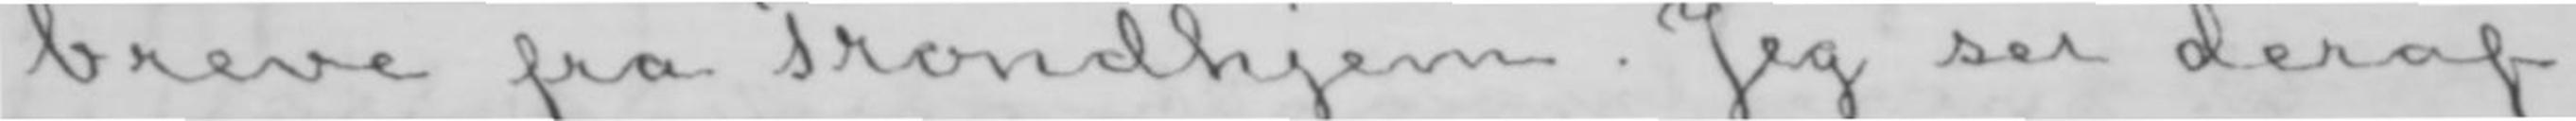breve fra Trondhjem. Jeg ser deraf
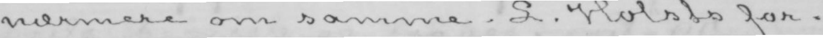nærmere om samme. L. Holsts for-
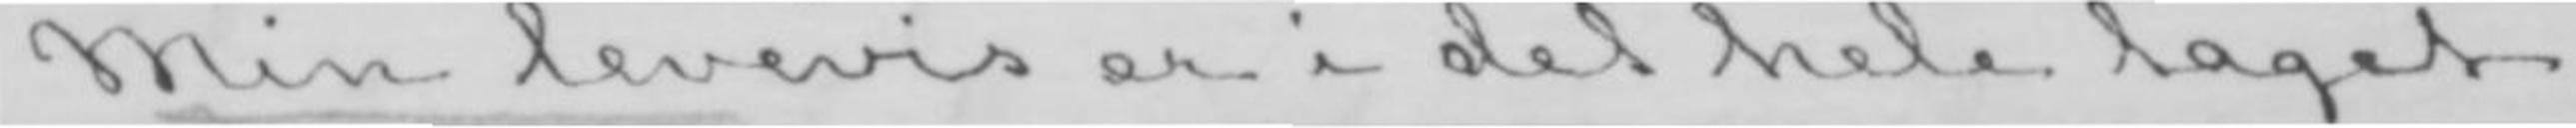Min levevis er i det hele taget
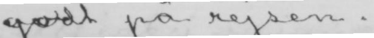godt på rejsen.
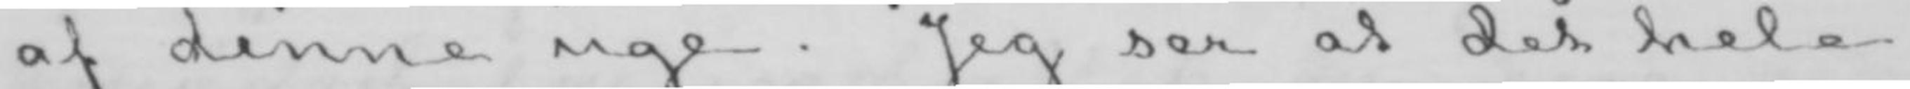af denne uge. Jeg ser at det hele
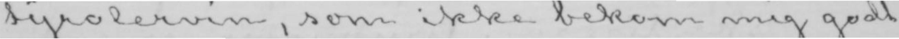tyrolervin, som ikke bekom mig godt,
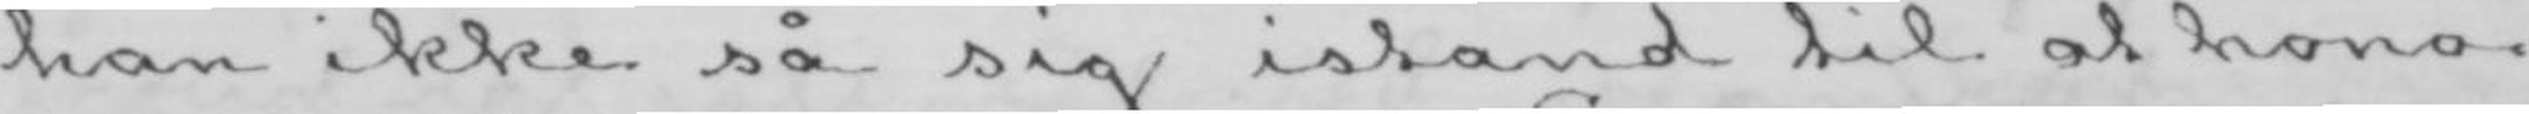han ikke så sig istand til at hono-
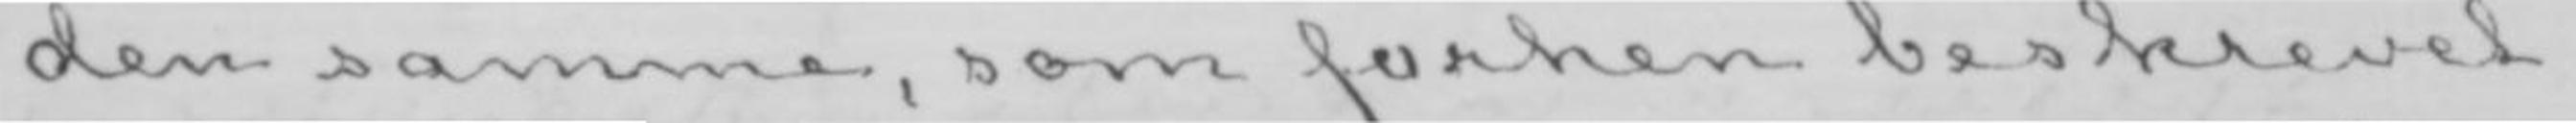den samme, som forhen beskrevet,
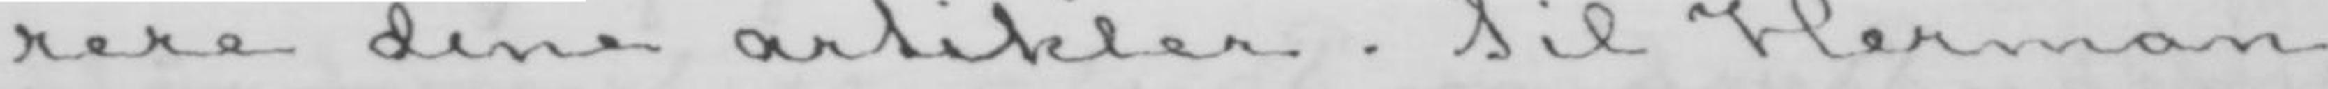rere dine artikler. Til Herman
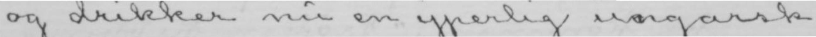og drikker nu en yperlig ungarsk
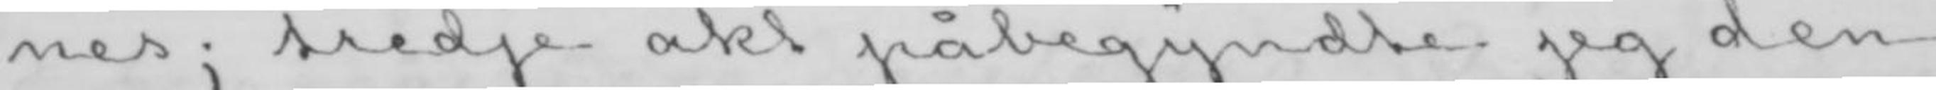nes; tredje akt påbegyndte jeg den
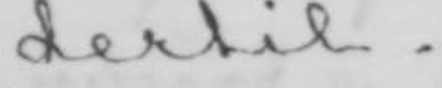dertil.
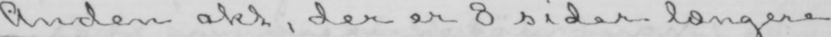Anden akt, der er 8 sider længere
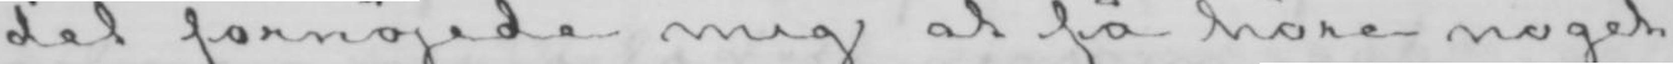det fornøjede mig at få høre noget
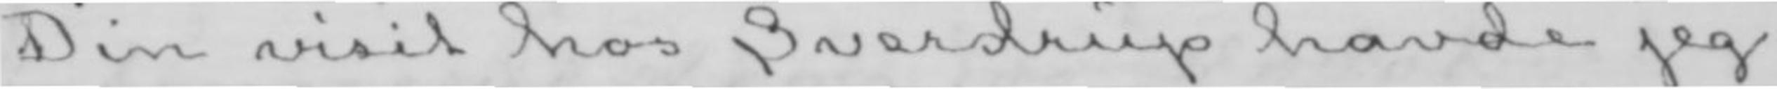Din visit hos Sverdrup havde jeg
